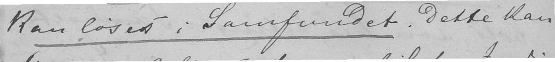kan løses i Samfundet. Dette kan
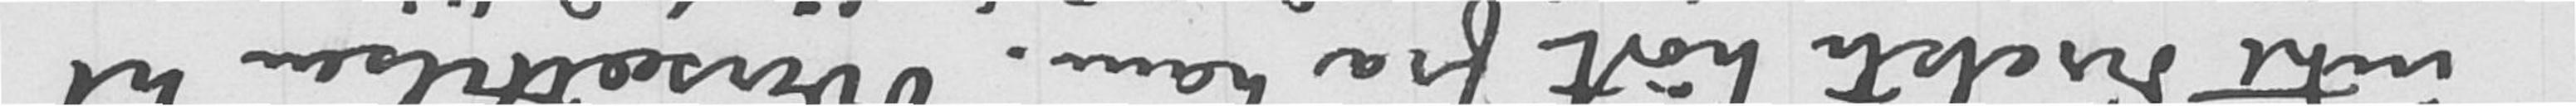intet direkte hørt fra ham. Oversættelsen til
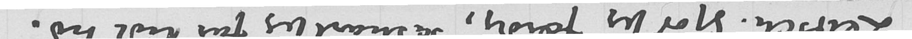Zeitsch. gjør jeg færdig, såsnart jeg får lidt tid.
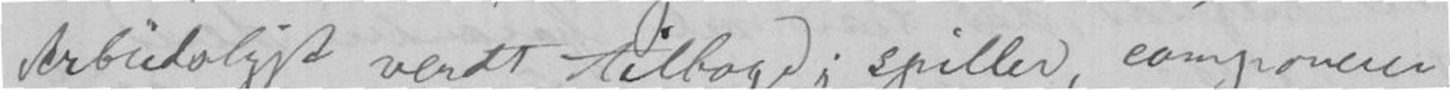Arbeidslyst vendt tilbage; spiller, componerer,
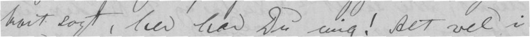havt sagt, her har Du mig! Alt vel i
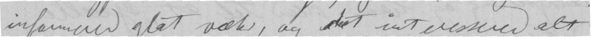informerer glat væk, og det interesserer alt
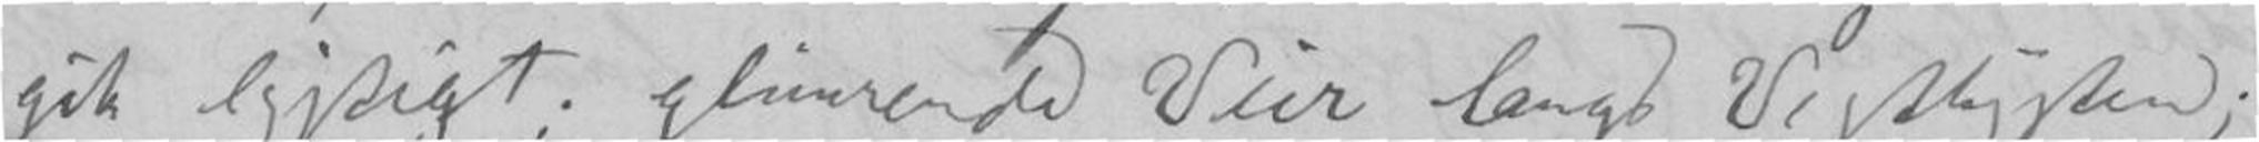gik lystigt; glimrende Veir langs Vestkysten;
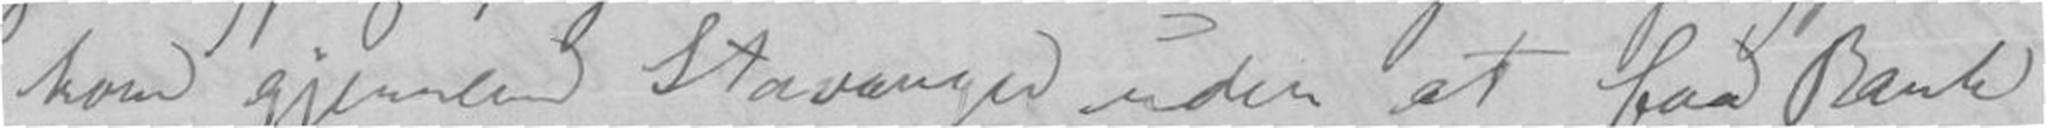kom gjennem Stavanger uden at faa Bank
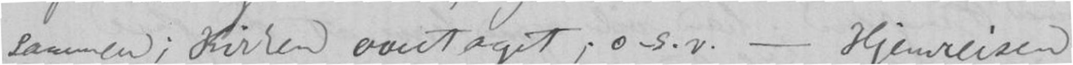sammen; Kirken overtaget; o.s.v. - Hjemreisen
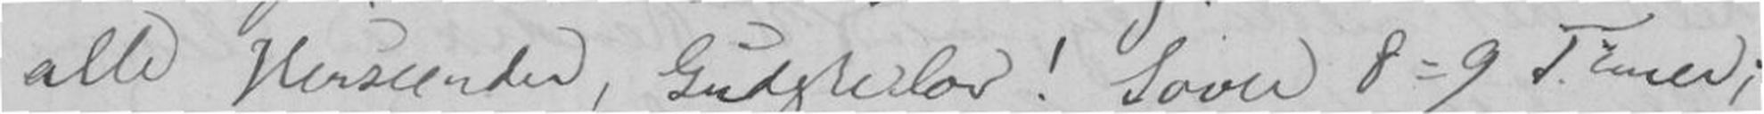alle Henseender, Gudskelov! Sover 8=9 Timer;
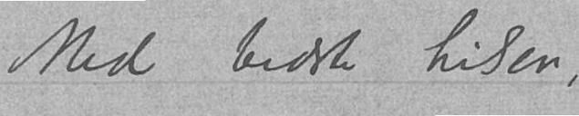Med bedste hilsen,
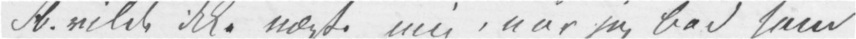Ib. vilde ikke nægte mig, når jeg bad ham
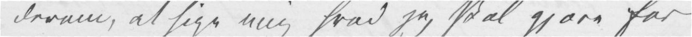derom, at sige mig hvad jeg skal gjøre for
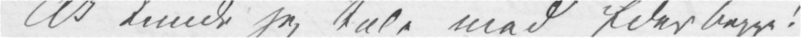Ak kunde jeg tale med Eder begge!
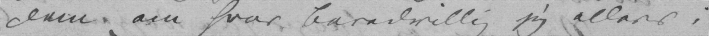Dem om hvor beredvillig jeg ellers i
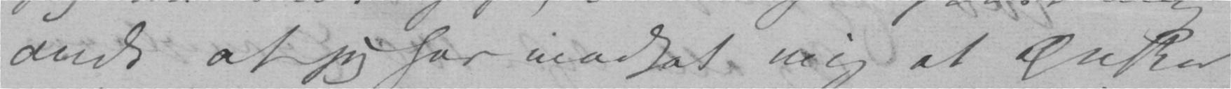ondt at jeg har modsat mig et Ønske
